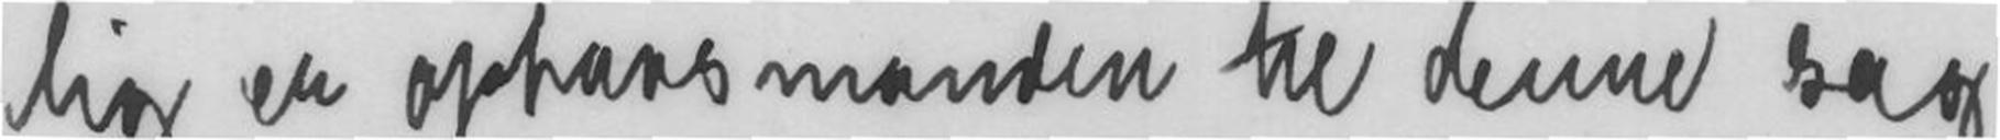tlig er ophavsmanden til denne sag,
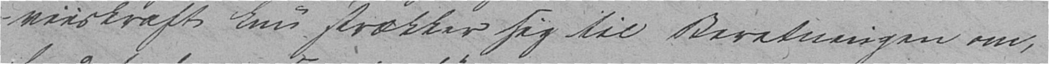viiskraft kun strækker sig til Beretningen om,
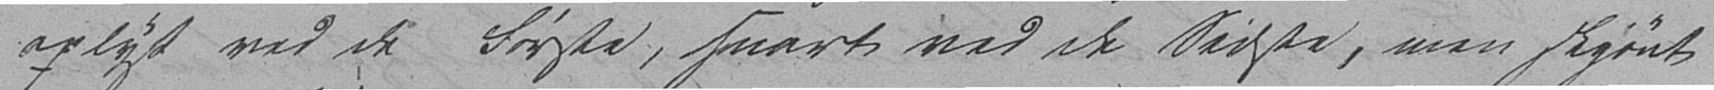oplyst ved de Første, snart ved de Sidste, men skjønt
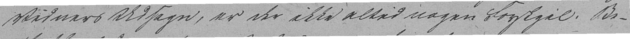Vidners Udsagn, er der ikke altid nogen Forskjel. Be-
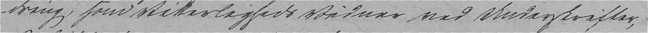dring, som Vitterligheds Vidner ved Underskrifter,
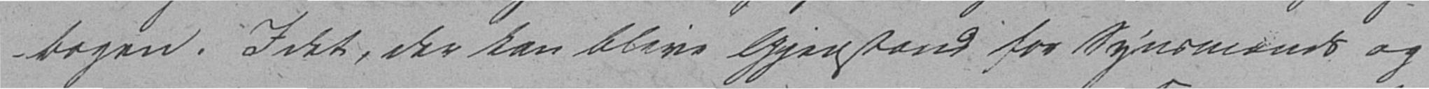bogen. Idet, der kan blive Gjenstand for Synsmænds og
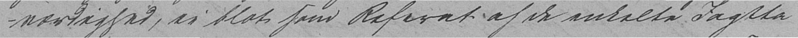værdighed, ei blot som Referat af de enkelte Iagtta-
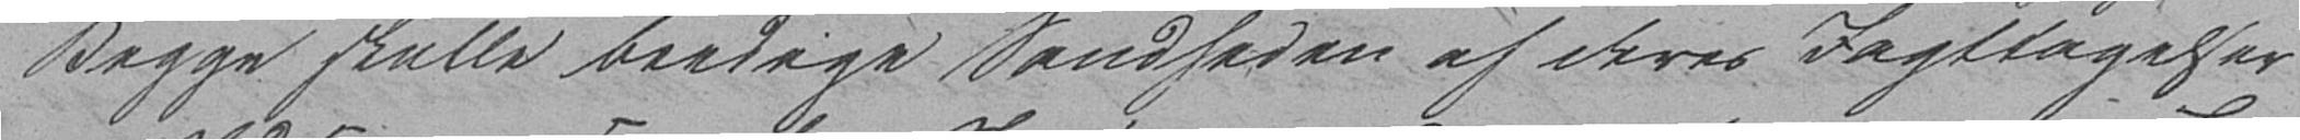Begge skulle beedige Sandheden af Deres Iagttagelser
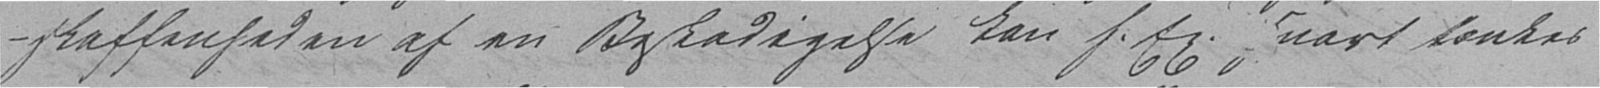skaffenheden af en Beskadigelse kan f. Ex snart tænkes
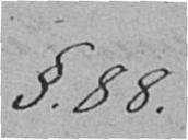§. 88.
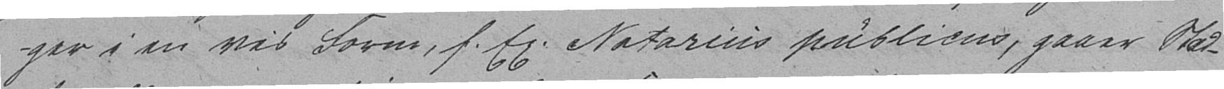ger i en vis Form, f. Ex. Notarius publicus, gaaer Stad-
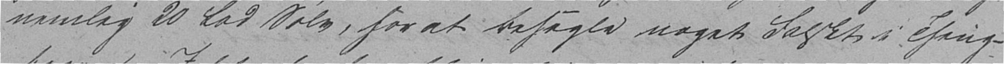nemlig 20 Lod Sølv, for at besegle noget falskt i Thing-
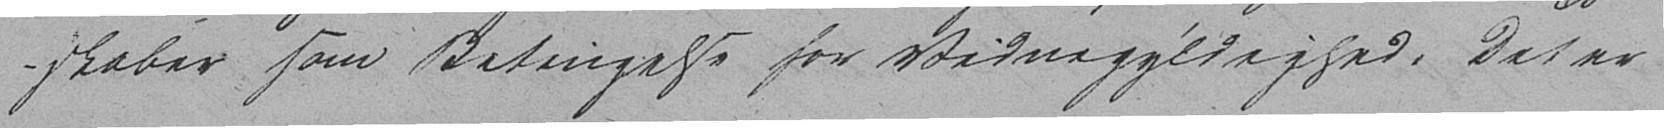skaber som Betingelse for Vidnegyldighed. Det er
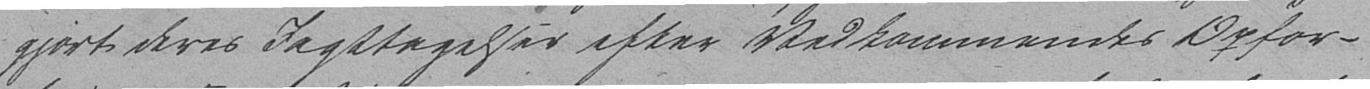gjort Deres Iagttagelser efter Vedkommendes Opfor-
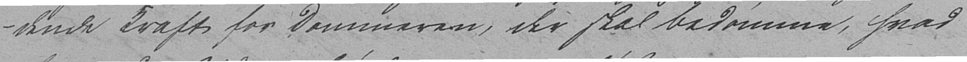dende Kraft for Dommeren, der skal bedømme, hvad
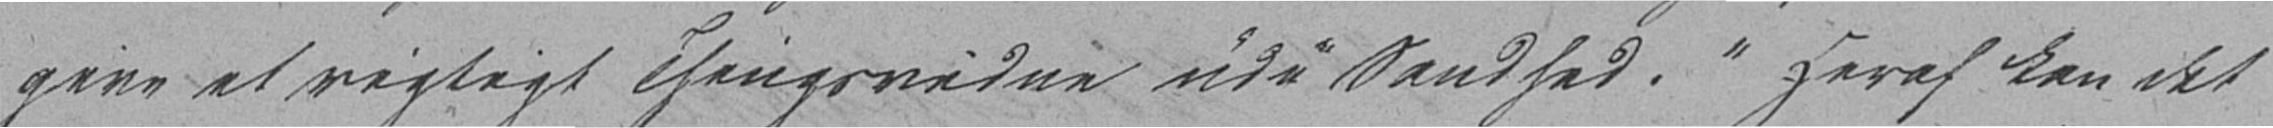give et rigtigt Thingsvidne udi Sandhed." Heraf kan det
